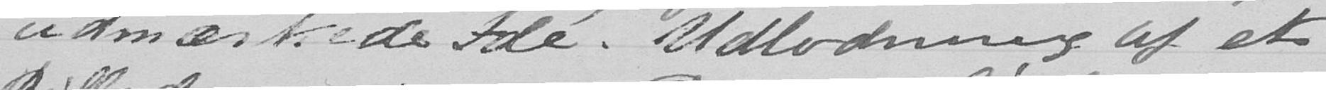udmærtede Ide. Udlodning af et
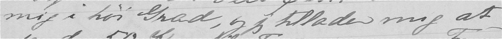mig i høi Grad, og jeg tillader mig at
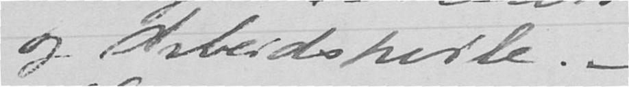og Arbeidshvile. -
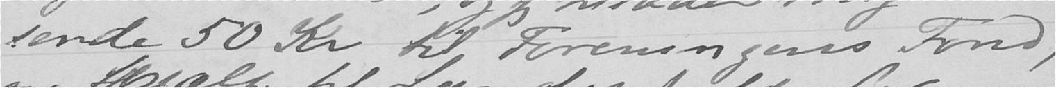sende 50 Kr til Foreningens Fond
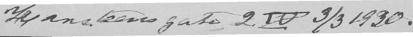Hansteens gate 2 IV 3/3 1930.
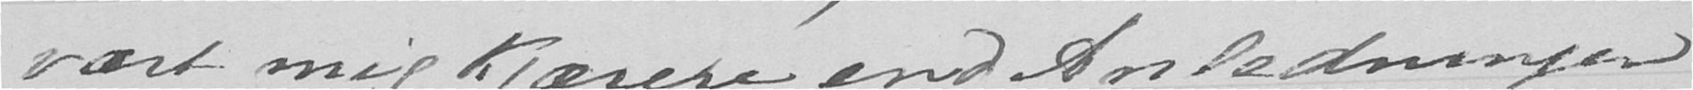vært mig kjærere end Anledningen
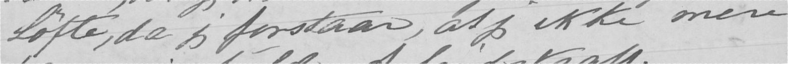Løfte, da jeg forstaar, at jeg ikke mere
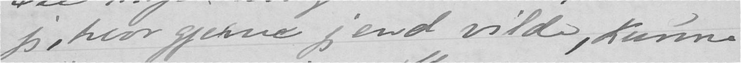jeg, hvor gjerne jeg end vilde, kunne
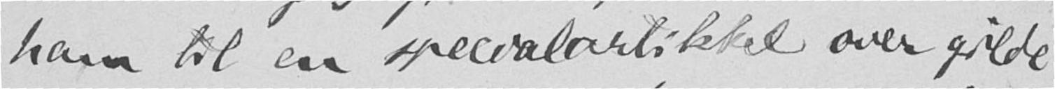ham til en specialartikkel over gilde-
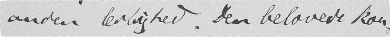anden leilighed. Den belovede kor-
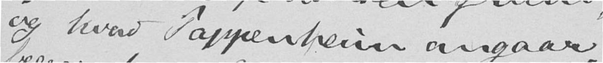og hvad Pappenheim angaar,
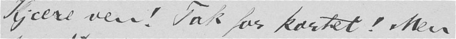Kjære ven! Tak for kortet! Men
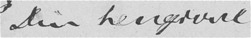Din hengivne
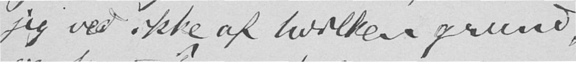jeg ved ikke af hvilken grund,
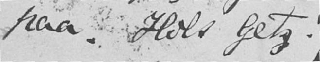paa. Hils Getz!
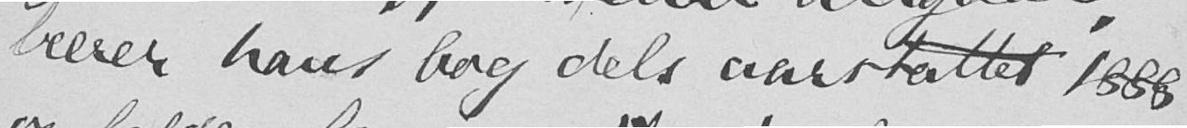bærer hans bog dels aarstallet 1888
